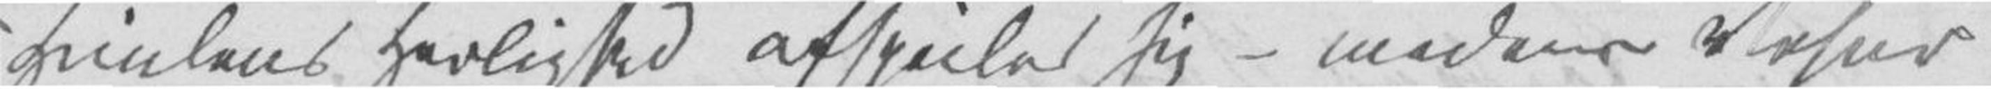Himlens Herlighed afspeiler sig – medens Vesuv
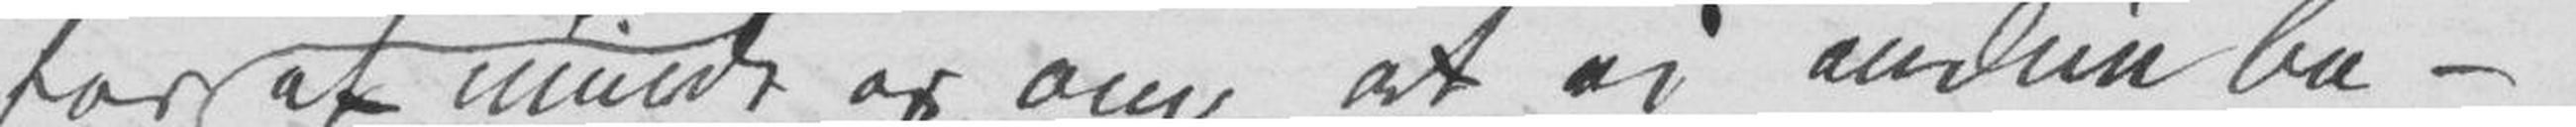for at minde os om at vi endnu be¬
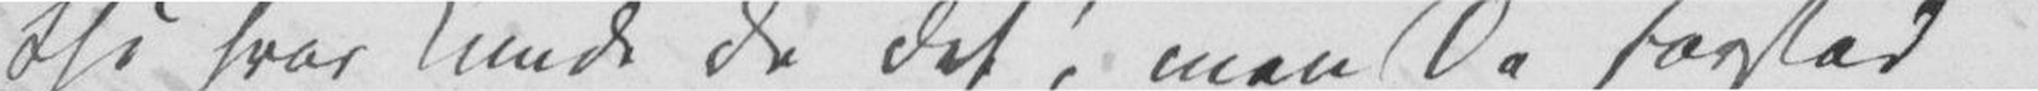thi hvor kunde de det! men De forstod
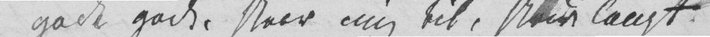godt godt, skriv mig til, skriv langt
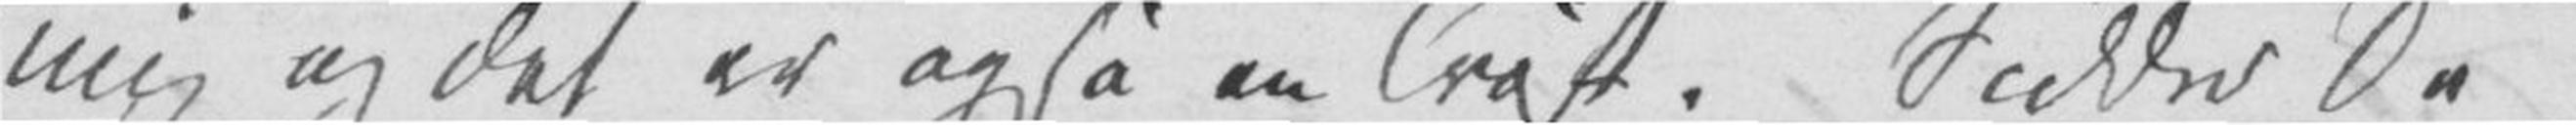mig og det er også en Trøst. Sidder De
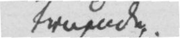truende
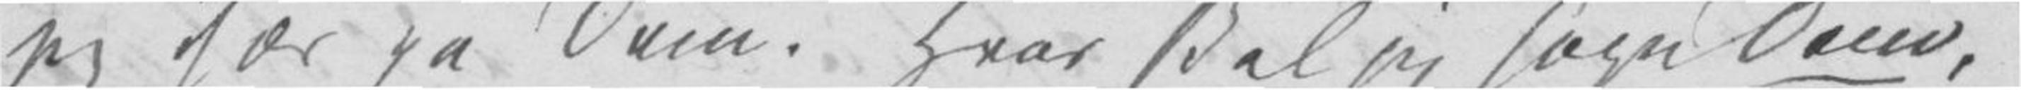jeg især på Dem. Hvor skal jeg søge Dem,
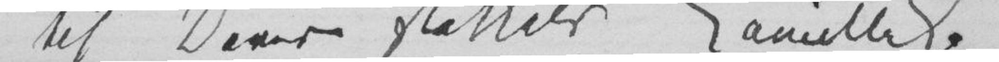til Deres stakkels Camilla C.
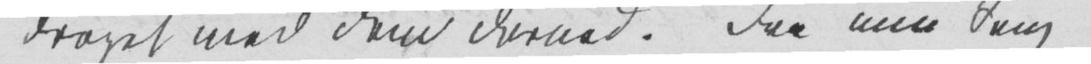draget med Dem derned! Fra min Seng
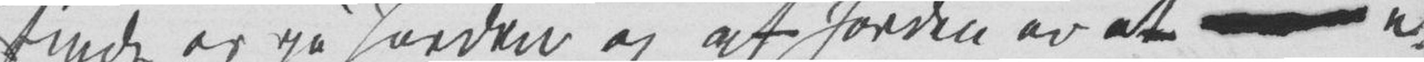finde os på Jorden og at Jorden er et –<gap />
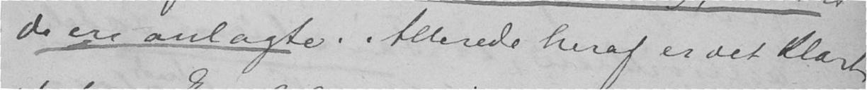de ere anlagte. Allerede heraf er det klart
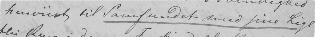henvist til Samfundet med sine Lige;
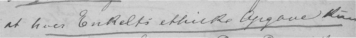at hver Enkelts ethiske Opgave kun
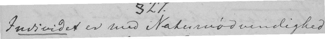Individet er med Naturnødvendighed
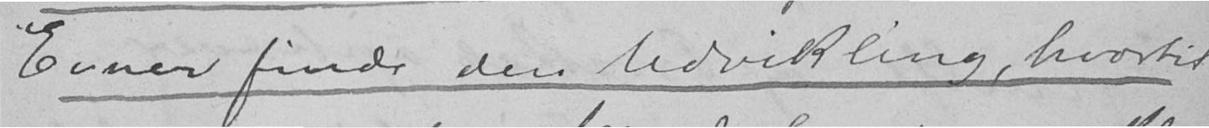Evner finde den Udvikling, hvortil
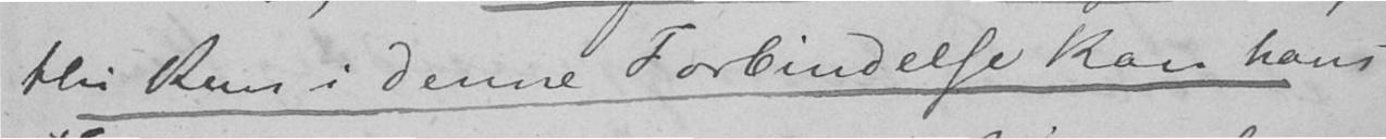thi kun i denne Forbindelse kan hans
